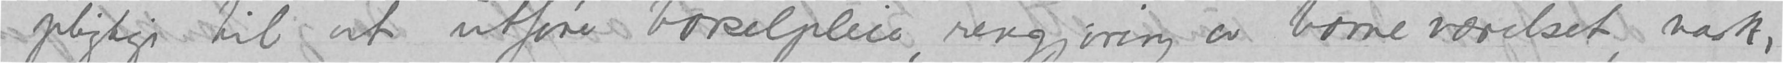pligtige til at utføre barselpleie, rengjøring av barneværelset, vask,
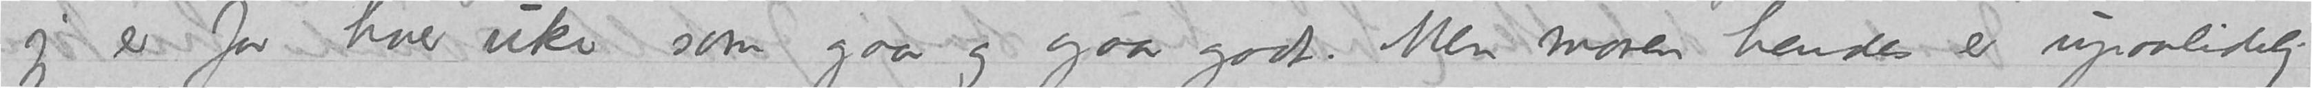jeg er for hver uke som gaar og gaar godt. Men maven hendes er upaalidelig
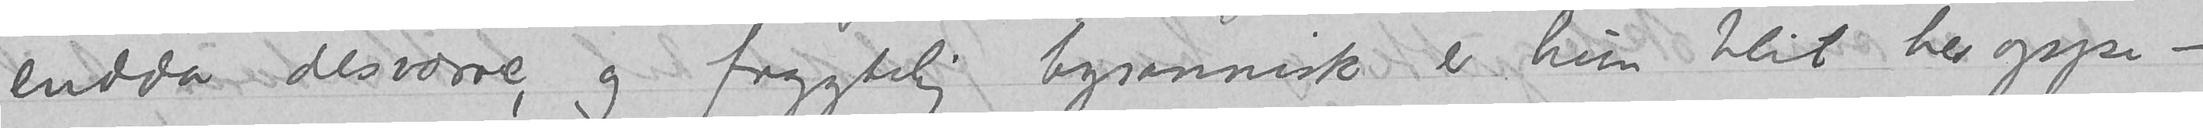endda desværre, og frygtelig tyrannisk er hun blit her oppe –
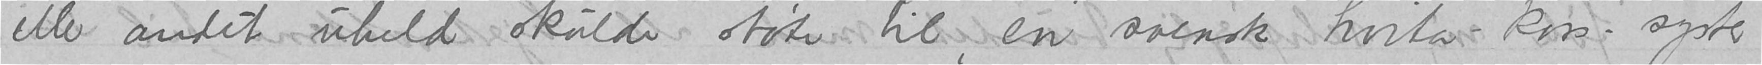eller andet uheld skulde støte til, en svensk hvita-kors-syster,
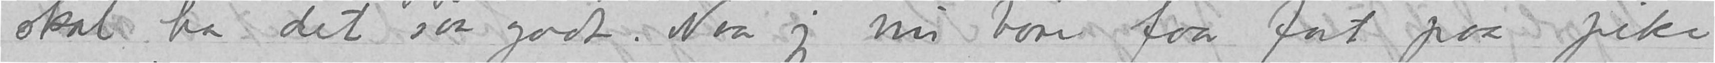skal ha det saa godt. Naar jeg nu bare faar fat paa pike
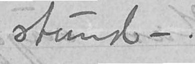stund-.
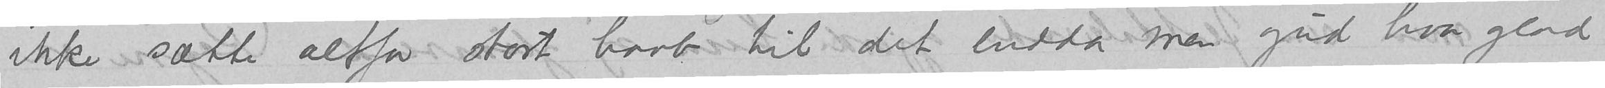ikke sætte altfor stort haab til det endda men gud hvor glad
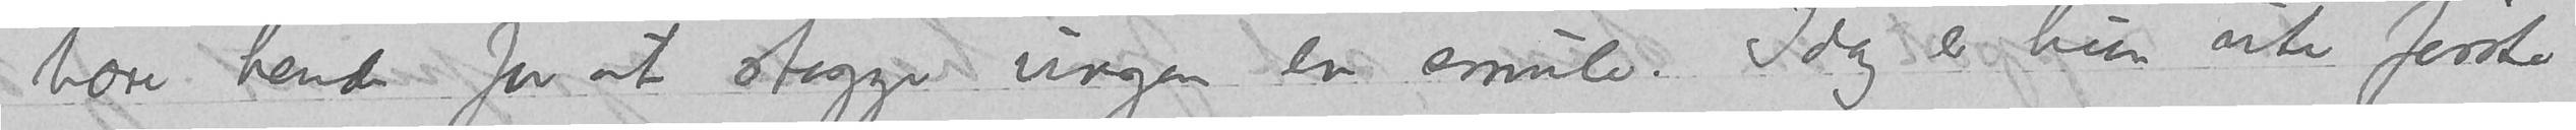bære hende for at stagge ungen en smule. Idag er hun ute første
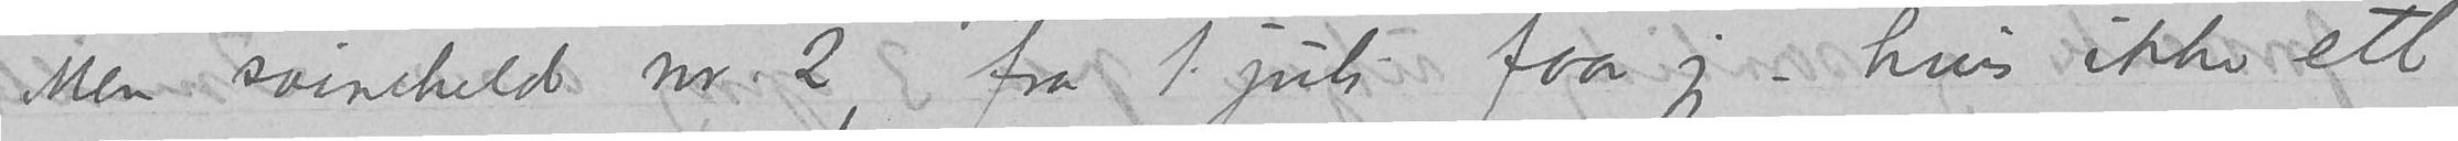Men svineheld nr. 2, fra 1. juli faar jeg – hvis ikke ett
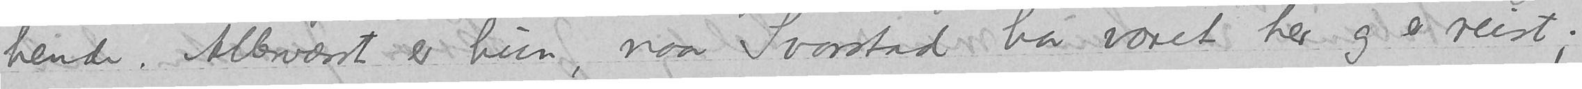hende. Allerværst er hun, naar Svarstad har været her og er reist;
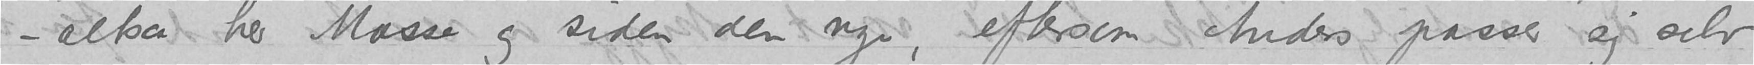familien – altsaa her Mosse og siden den nye, eftersom Anders passer sig selv
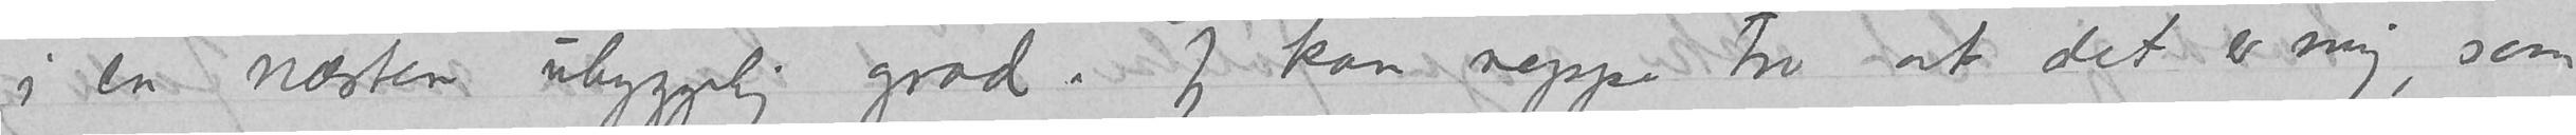i en næsten  uhyggelig grad. Jeg kan neppe tro at det er mig, som
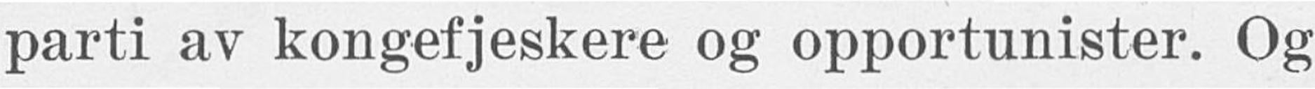parti av kongefjeskere og opportunister. Og
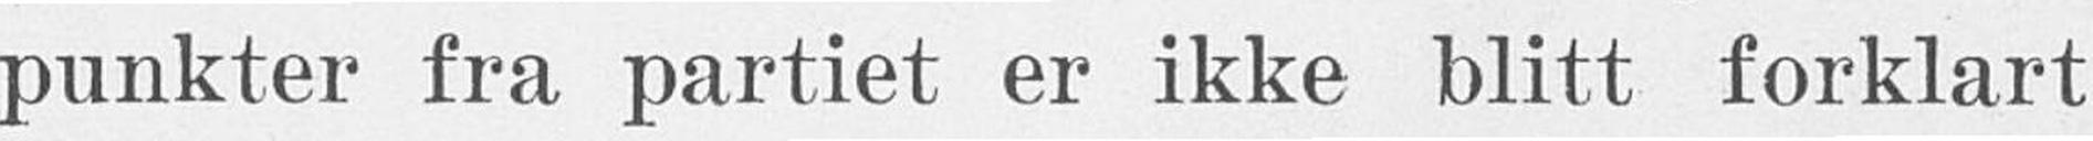punkter fra partiet er ikke blitt forklart
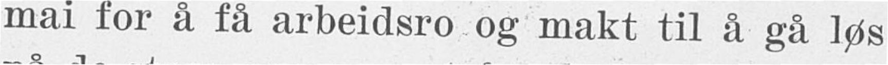mai for å få arbeidsro og makt til å gå løs
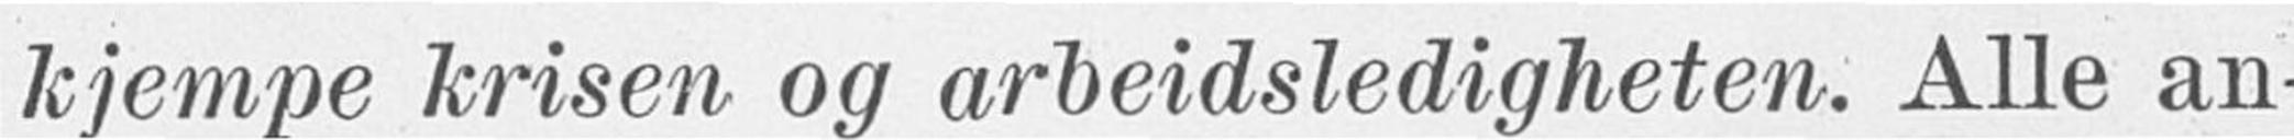kjempe krisen og arbeidsledigheten. Alle an
